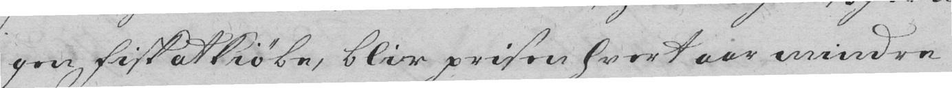gen Fisk at kiøbe, blir prisen hvert aar mindre
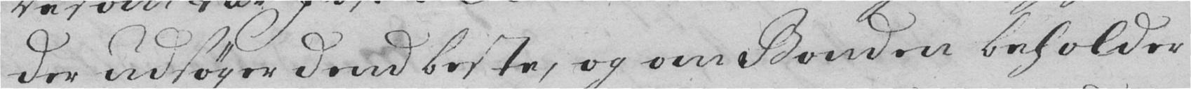der udsøger dend beste, og om Bonden beholder
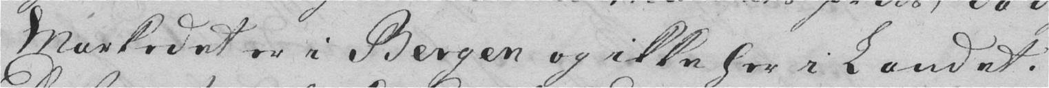Markedet er i Bergen og ikke her i Landet.
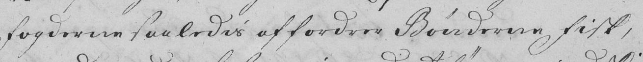Fogderne saaledis affordrer Bønderne Fisk,
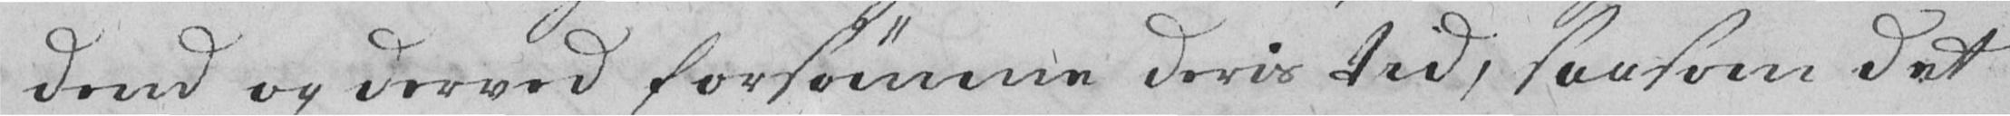dend og derved forsømme deris tid, saasom det
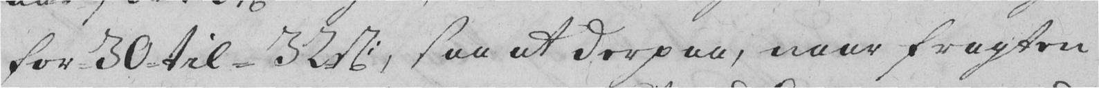for 30 til 32 sk., saa at derpaa, naar fragten
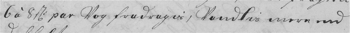6 á 8 sk. par Vog fradragis, Vandtis mere end
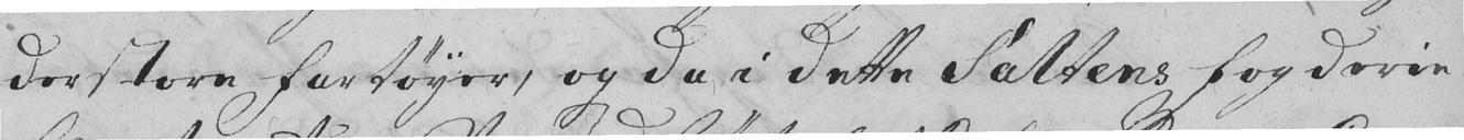der store Fartøyer, og da i dette Saltens Fogderie
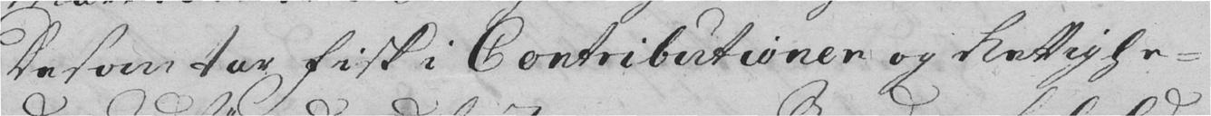De som tar fisk i Contributioner og Rettighe-
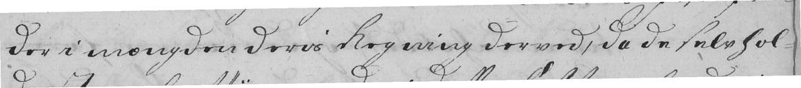der i mængden deris Regning derved, da de selv hol-
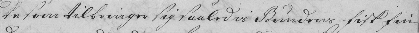De som tilbringer sig saaledis Bundens Fisk fin-
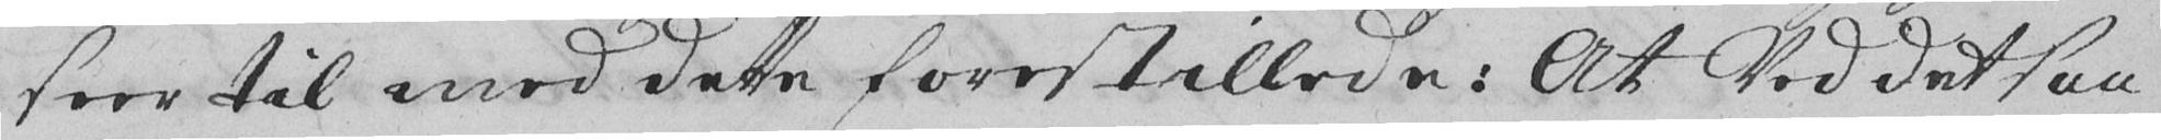seer til med dette forestillede: At Ved det saa
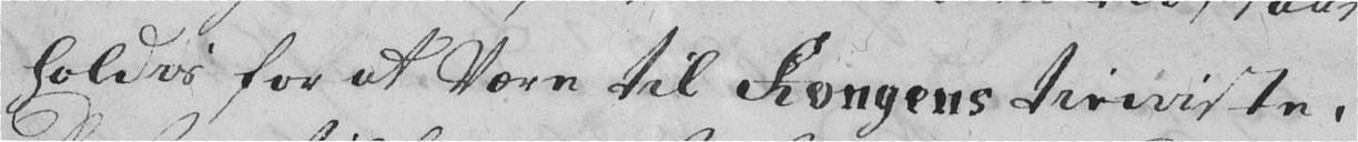holdis for at Være til Kongens tieniste.
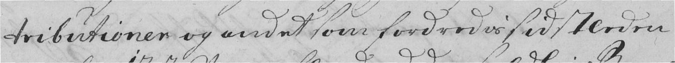tributioner og andet som fordredis sidstleden
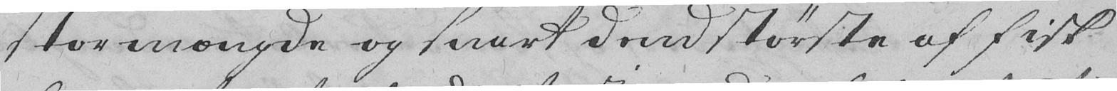stor mængde og snart dend største af fisk
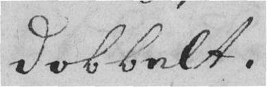dobbelt.
